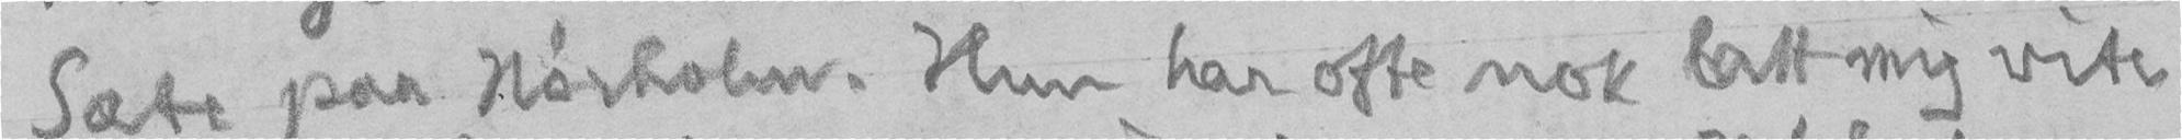Sæte paa Nørholm. Hun har ofte nok latt mig vite
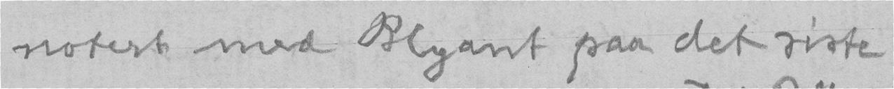notert med Blyant paa det siste
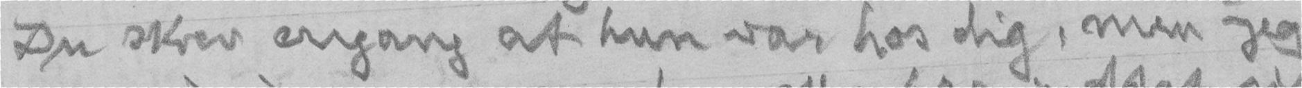Du skrev engang at hun var hos dig, men jeg
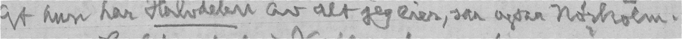at hun har Halvdelen av alt jeg eier, saa ogsaa Nørholm.
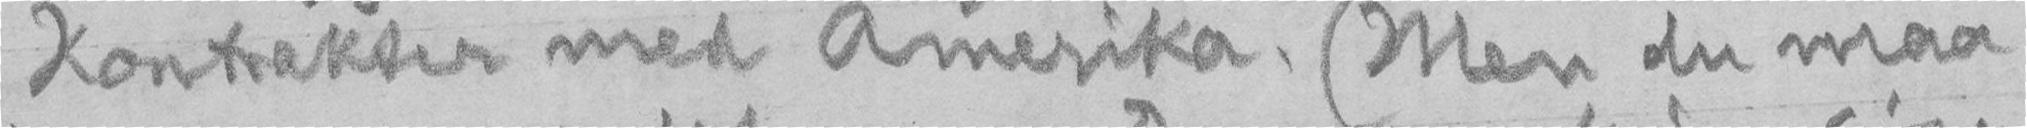Kontrakter med Amerika. (Men du maa
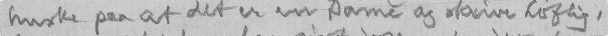huske paa at det er en Dame og skrive høflig,
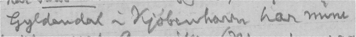Gyldendal i Kjøbenhavn har mine
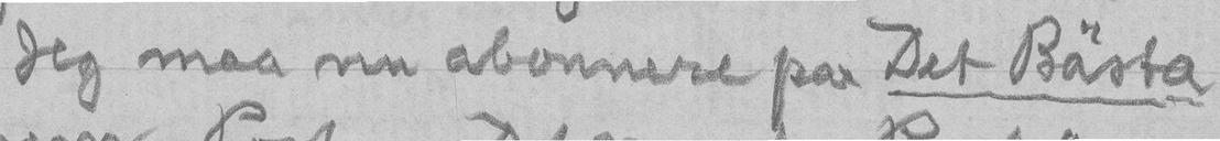Jeg maa nu abonnere paa Det Bästa
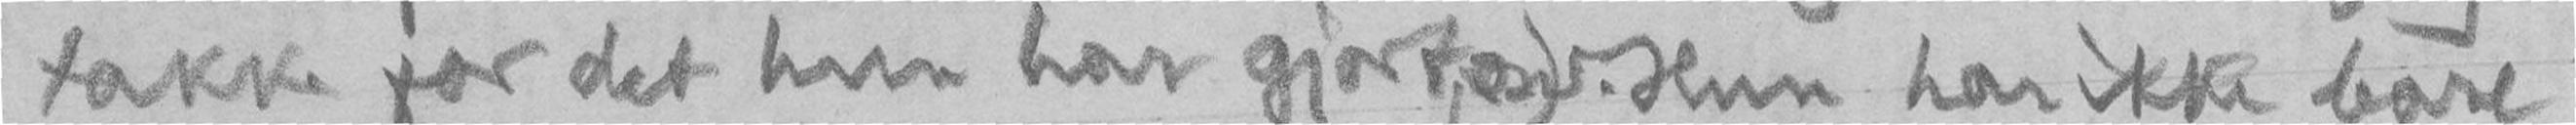takke for det hun har gjort os)v. Hun har ikke bare
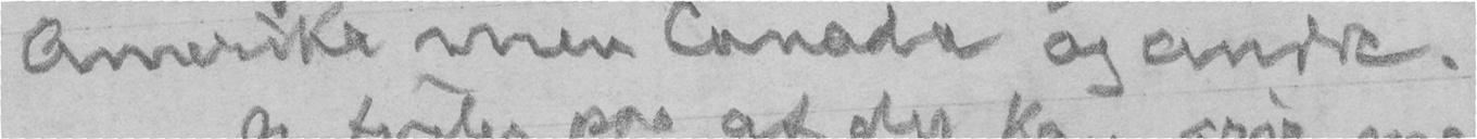Amerika men Canada og andre.
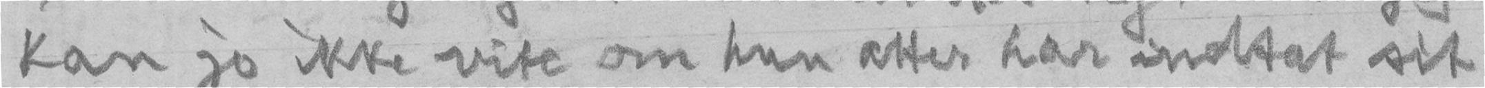kan jo ikke vite om hun atter har indtat sit
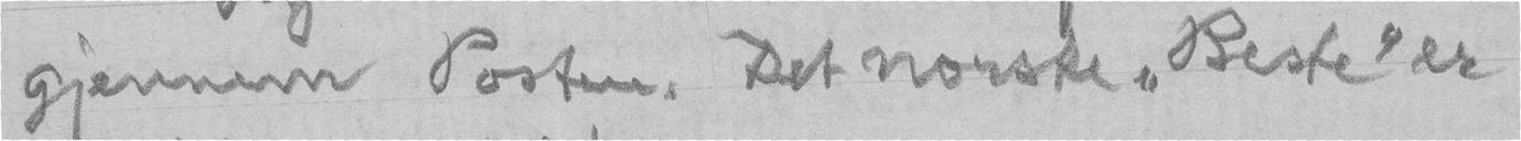gjennem Posten. Det norske "Beste" er
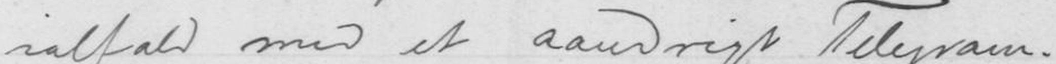ialfald med et aandrigt Telegram.
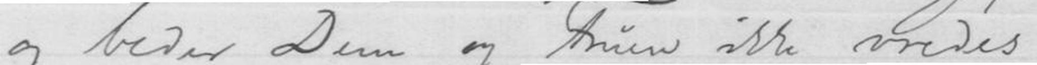og beder Dem og Fruen ikke vredes
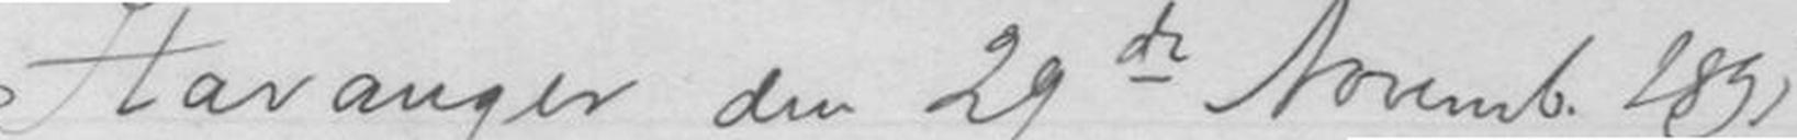Stavanger den 29de Novemb. 1891
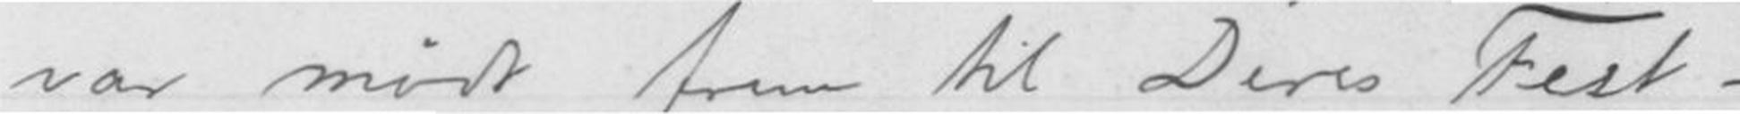var nødt frem til Deres Fest-
